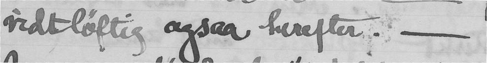vidtløftig ogsaa herefter. -
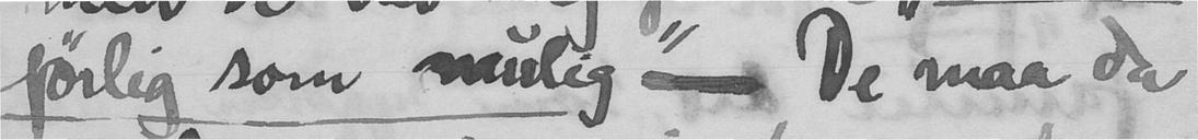førlig som mulig" - De maa da
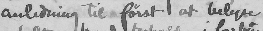anledning til først at belyse
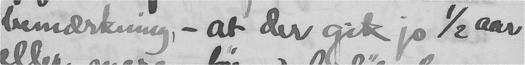bemærkning, - at der gik jo 1/2 aar
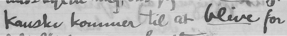kanske kommer til at blive for
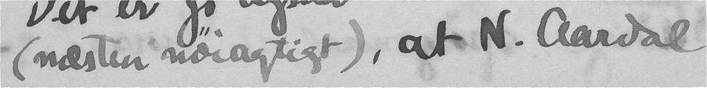(næsten nøiagtigt), at N. Aardal
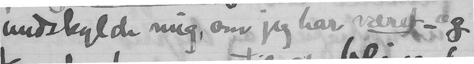undskylde mig, om jeg har været - og
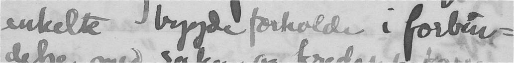enkelte bygde forholde i forbin-
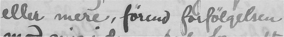eller mere, førend forfølgelsen
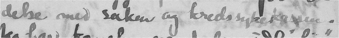delse med saken og kredssykekassen.
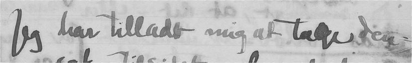Jeg har tilladt mig at tage den-
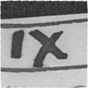IX
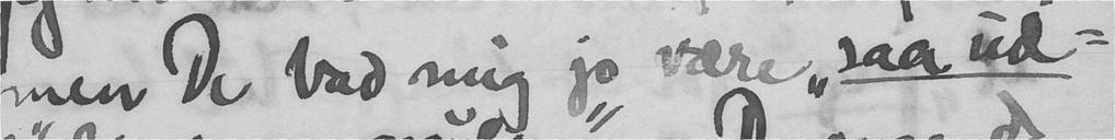men De bad mig jo være "saa ud-
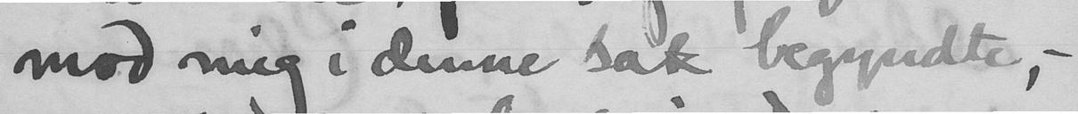mod mig i denne sak begyndte, -
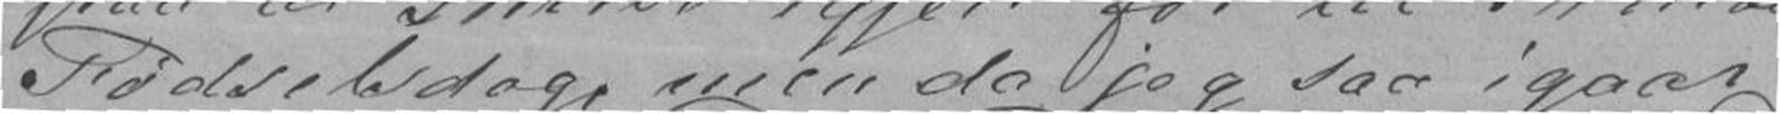Fødselsdag, men da jeg saa igaar
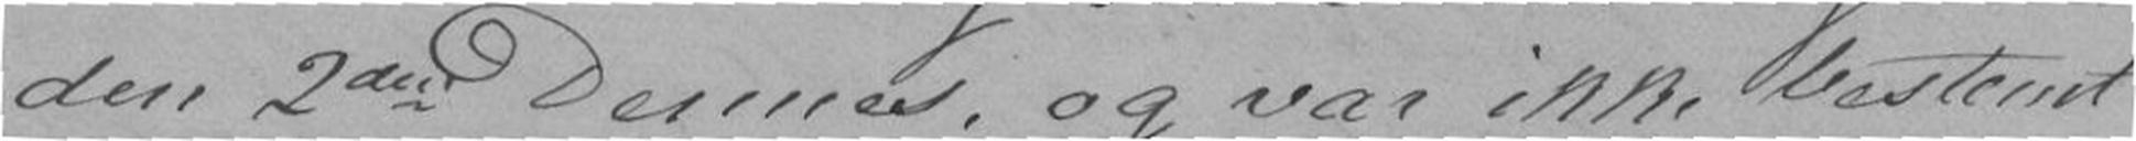den 2den Dennes, og var ikke bestemt
